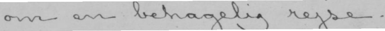om en behagelig rejse.
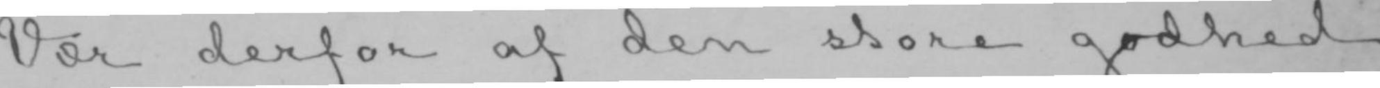Vær derfor af den store godhed
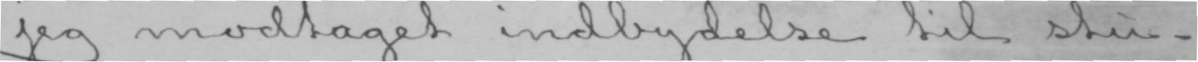jeg modtaget indbydelse til stu-
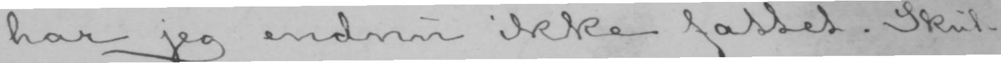har jeg endnu ikke fattet. Skul-
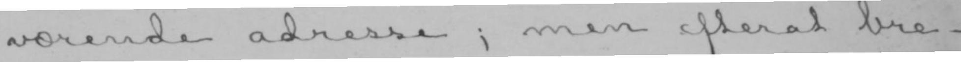værende adresse; men efterat bre-
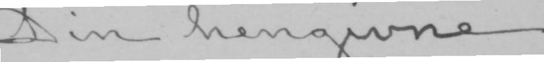Din hengivne
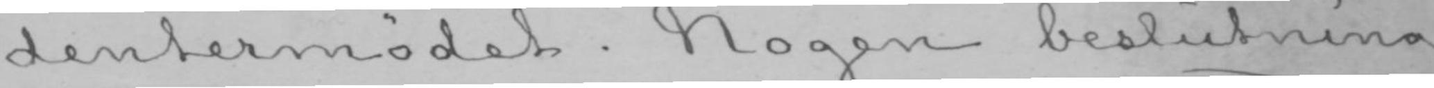dentermødet. Nogen beslutning
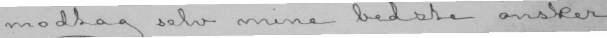modtag selv mine bedste ønsker
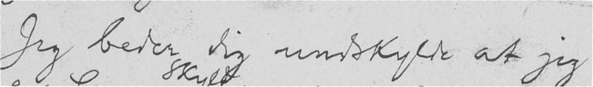Jeg beder dig undskylde at jeg
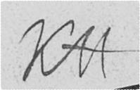KH
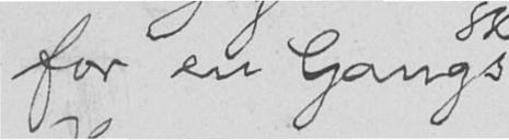for en Gangs
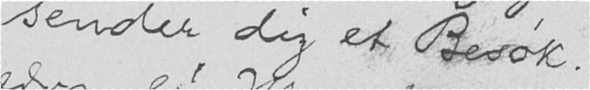sender dig et Besøk.
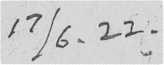17/6. 22.
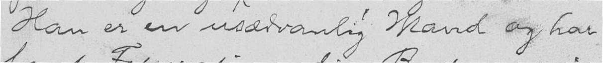Han er en usædvanlig Mand og har
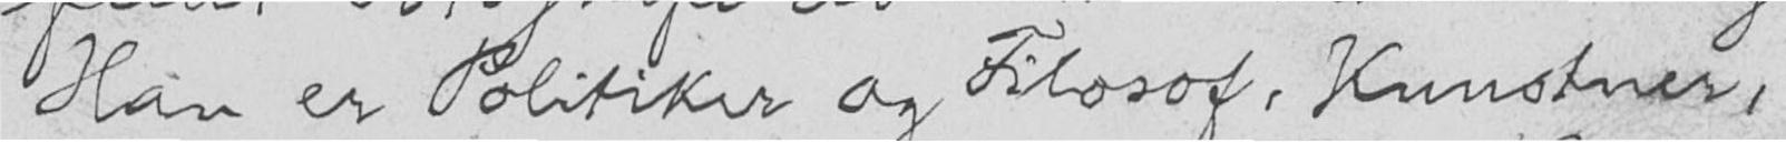Han er Politiker og Filosof, Kunstner,
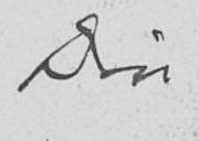Din
